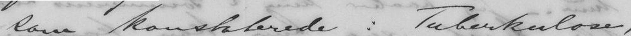om konstaterede: Tuberkulose,
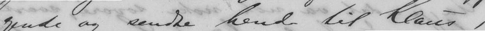gende og sendte hende til Klaus,
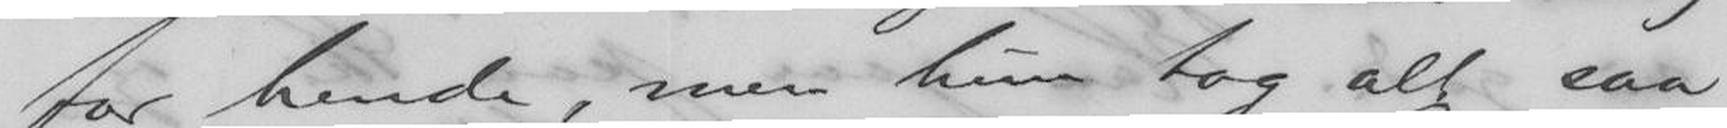for hende, men hun tog alt saa
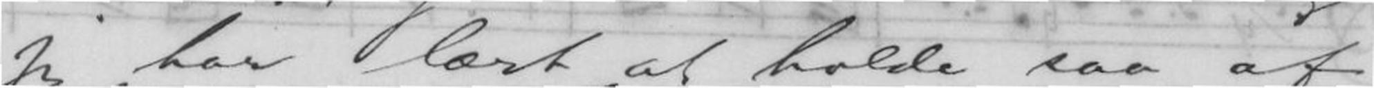jeg har lært at holde saa af,
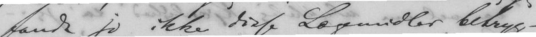fandt jo ikke disse Lægemidler betryg-
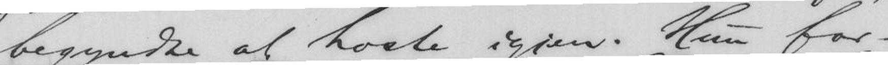begyndte at hoste igjen. Hun for-
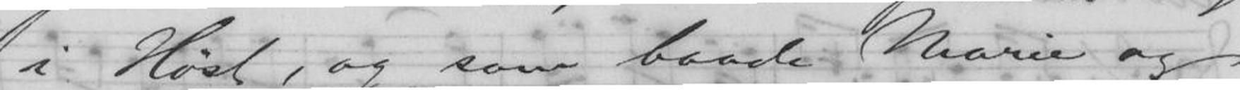i Høst, og som baade Marie og
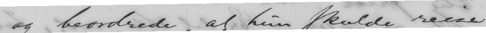og beordrede, at hun skulde reise
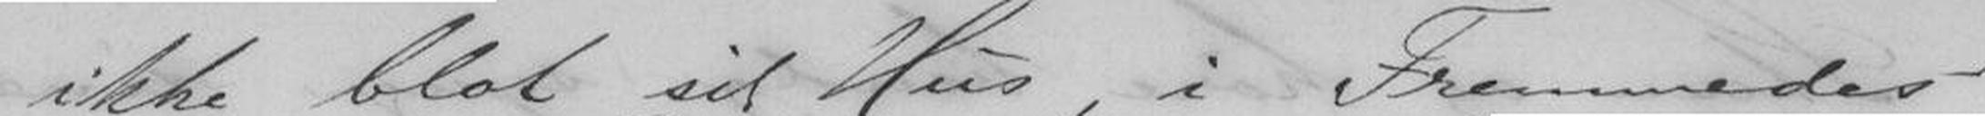ikke blot sit Hus, i Fremmedes
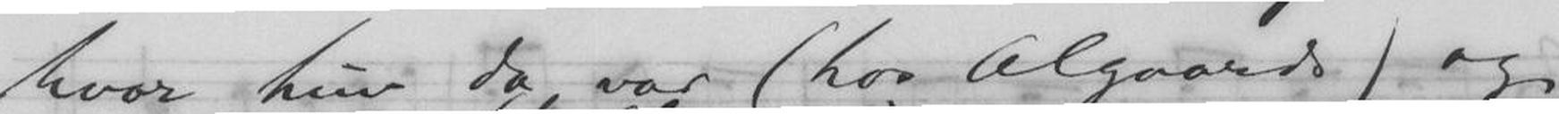hvor hun da var (hos Algaards) og
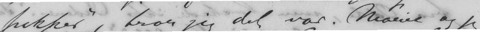sukker", tror jeg det var. Marie og jeg
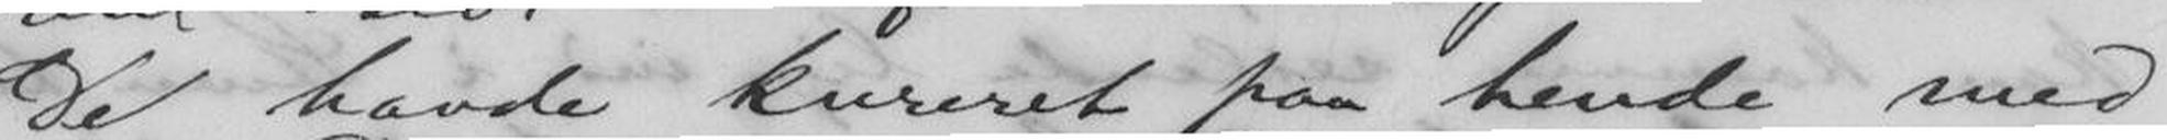De havde kureret paa hende med
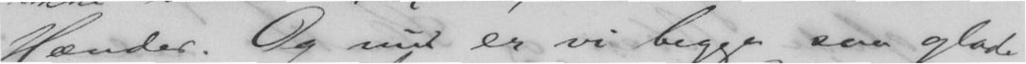Hænder. Og nu er vi begge saa glade
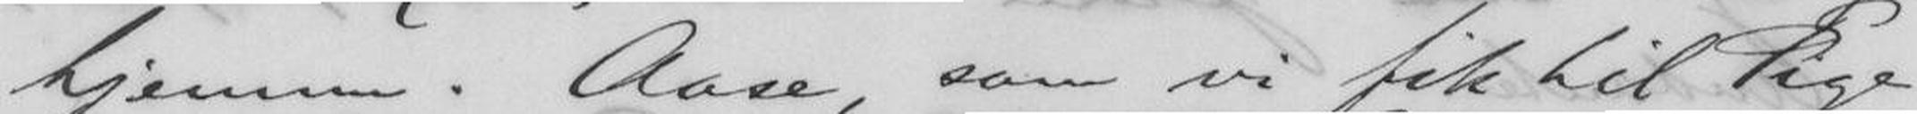hjemme. Aase, som vi fik til Pige
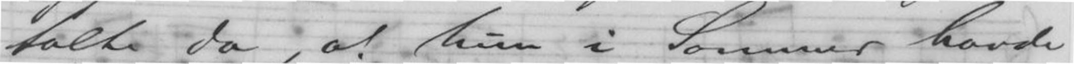talte da, at hun i Sommer havde
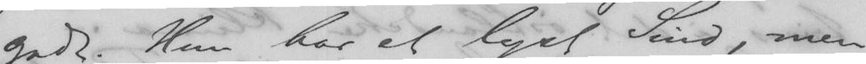godt. Hun har et lyst Sind, men
